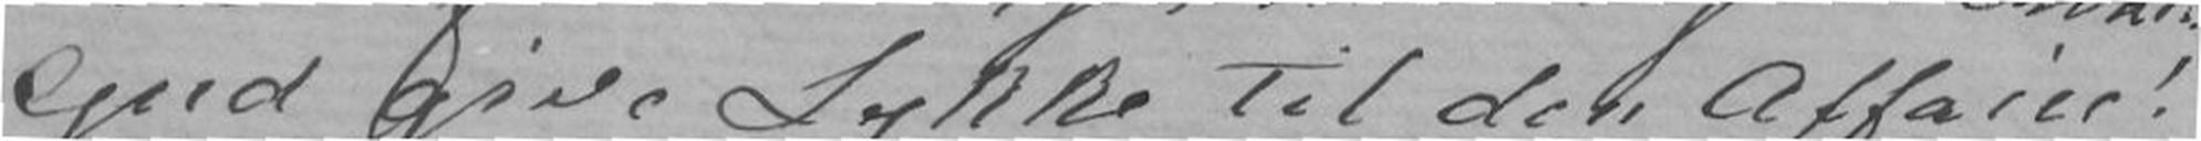Gud give Lykke til den Affaire!
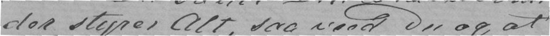der styrer Alt, saa veed Du og at
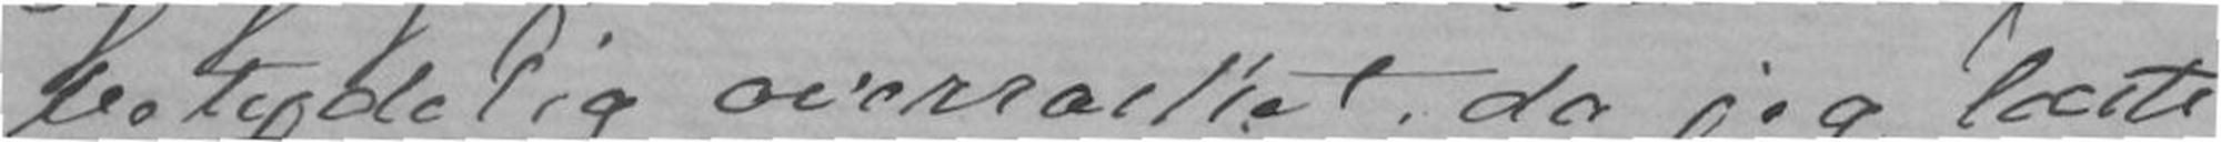betydelig overrasket, da jeg læste
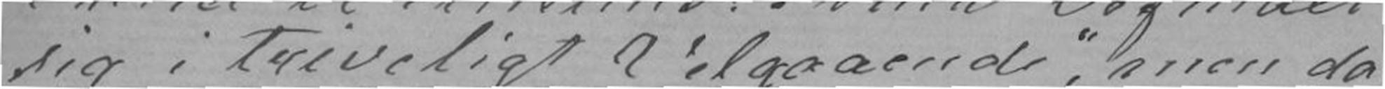sig i triveligt Velgaaende", men da
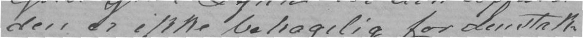den er ikke behagelig for den stak-
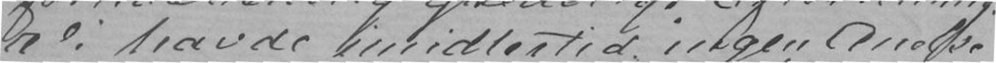Vi havde imidlertid ingen Anelse
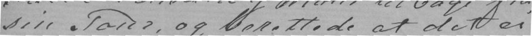sin Tour, og berettede at det ei
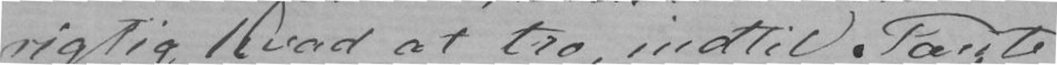rigtig hvad at tro, indtil Tante
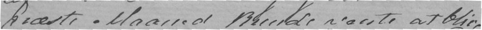næste Maaned kunde vænte at blive
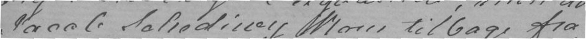Jacob Schediwy kom tilbage fra
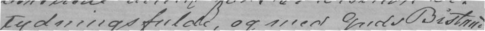tydningsfulde, og med Guds Bistand
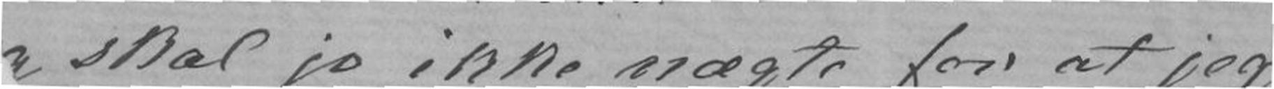skal jo ikke nægte for at jeg
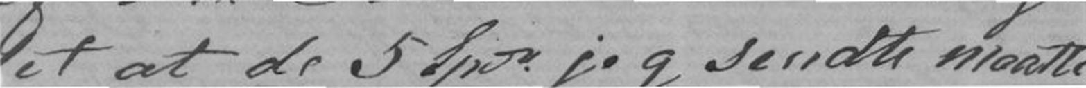at de 5Spdo. jeg sendte maatte
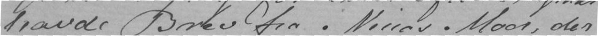havde Brev fra Ninas Moer, der
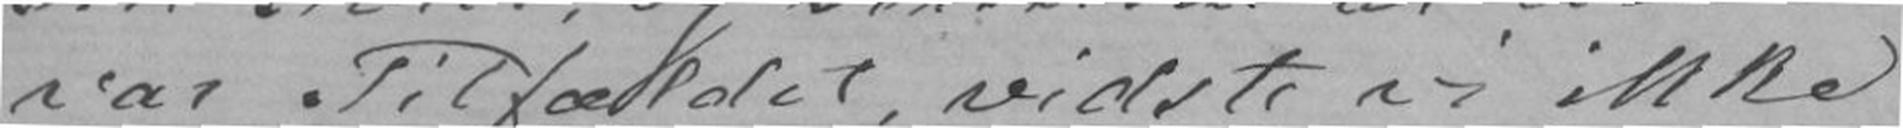var Tilfældet, vidste vi ikke
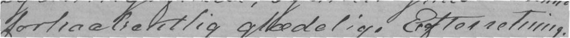forhaabentlig glædelige Efterretning.
